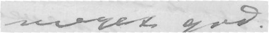meget god.
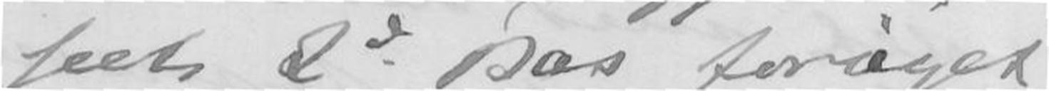seet 2d Bas forøget
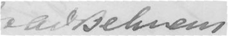Joh D Behrens
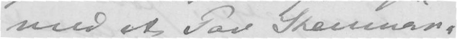med et Par Stemmer.
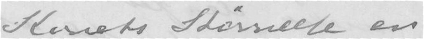Korets Størrelse er
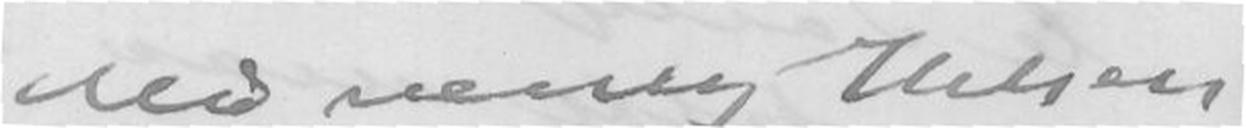Med venlig Hilsen
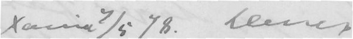Xania 7/5 78Deres
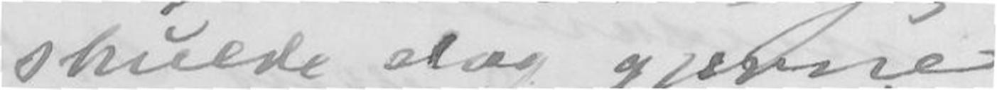skulde da gjerne
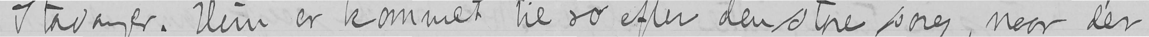Stavanger. Hun er kommet til ro efter den store sorg, naar der
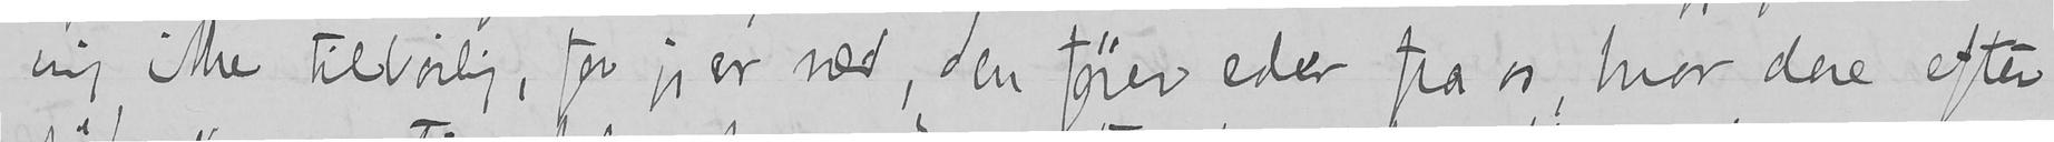mig ikke tilbørlig, for jeg er ræd, den fører eder fra os, hvor dere efter
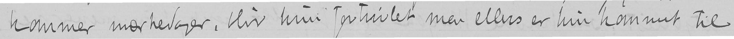kommer mærkedager, blir hun fortvivlet men ellers er hun kommet til
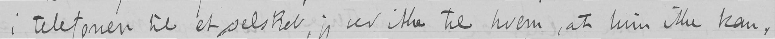i telefonen til et selskab, jeg ved ikke til hvem, at hun ikke kan,
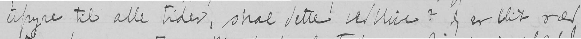uhyre til alle tider, skal dette vedblive? Jeg er blit ræd
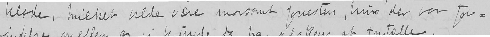klode, hvilket vilde være morsomt forresten, hvis der var for-
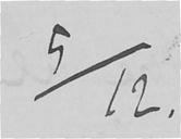5/12.
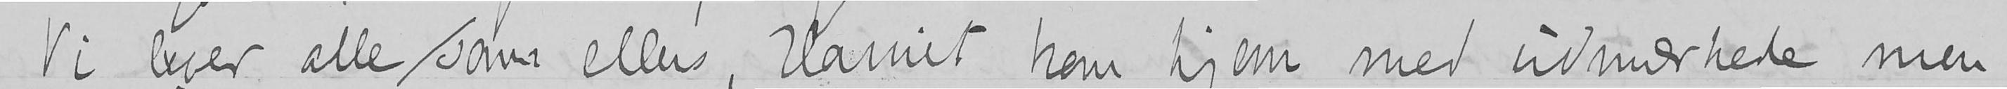Vi lever alle som ellers, Harriet kom hjem med udmærkede men
